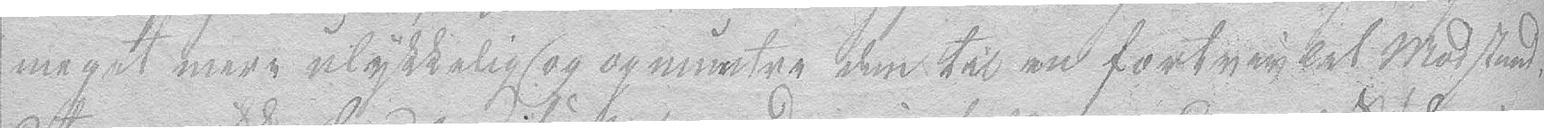meget mere ulykkelig og opmuntre dem til en fortvivlet Modstand.
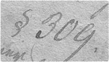§ 309.
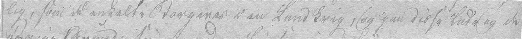lig, som de enkelte Borgeres i en Landkrig, og paa disse lade og de
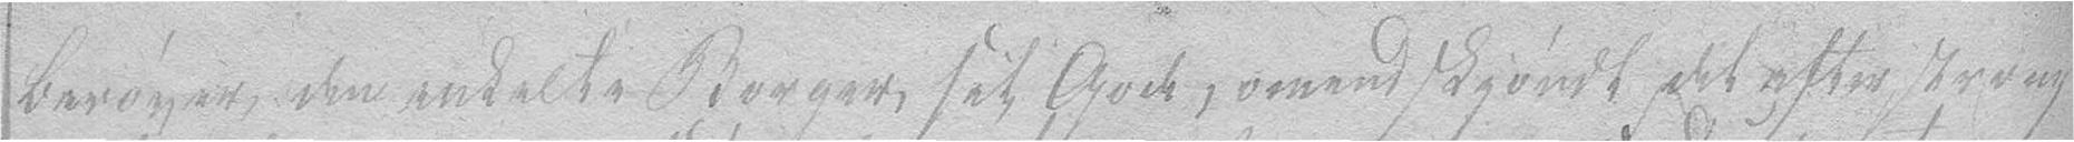berøver den enkelte Borger sit Gods, omendskjøndt det efter stræng
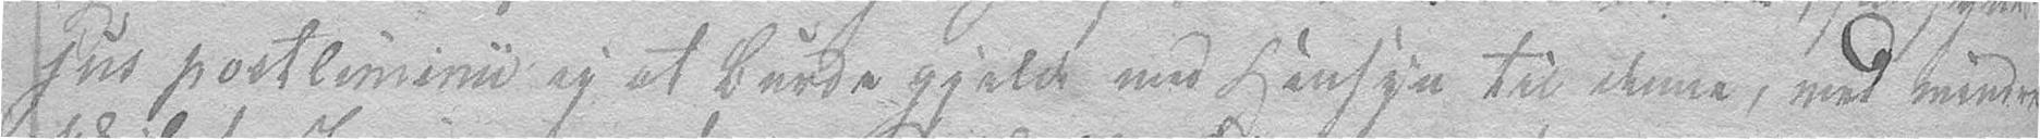jus postliminii ey at burde gjelde med Hensyn til denne, med mindre
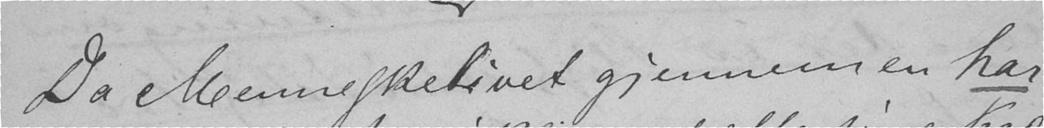Da Menneskelivet gjennem en har-
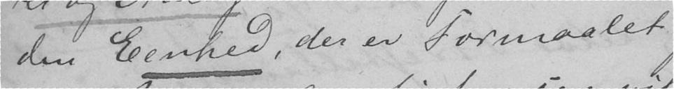den Eenhed, der er Formaalet
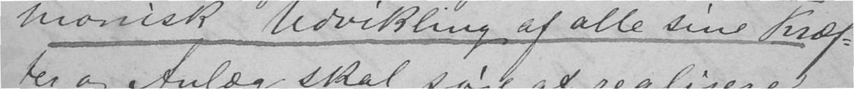monisk Udvikling af alle sine Kræf-
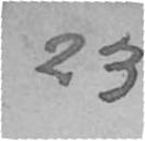23
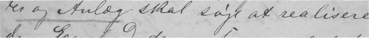ter og Anlæg skal søge at realisere
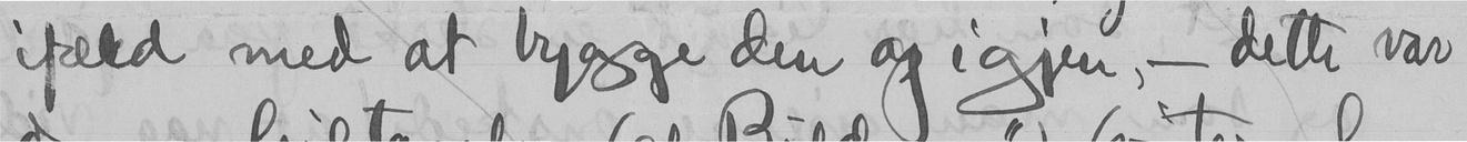ifæld med at bygge den op igjen, - dette var
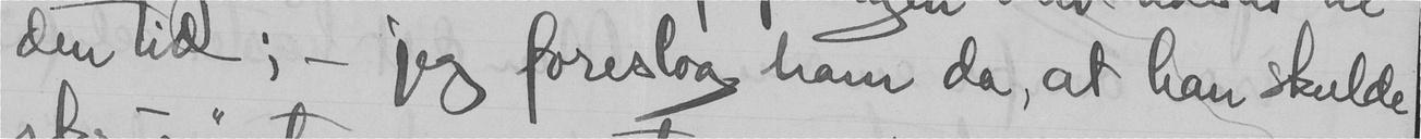den tid; - jeg foreslog ham da, at han skulde
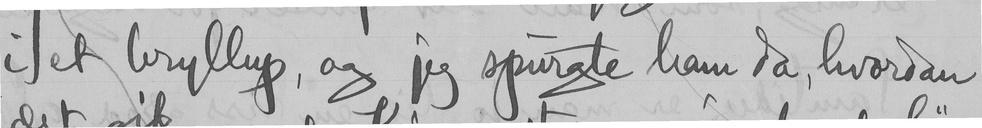i et bryllup, og jeg spurgte ham da, hvordan
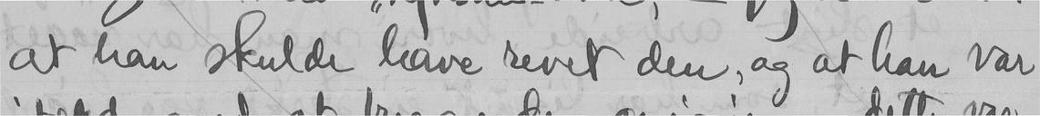at han skulde have revet den, og at han var
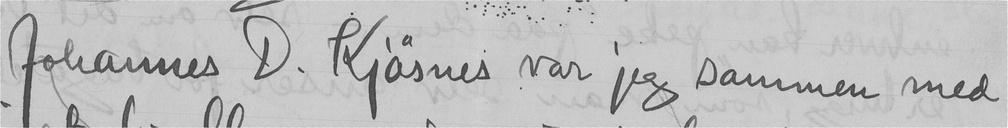Johannes D. Kjøsnes var jeg sammen med
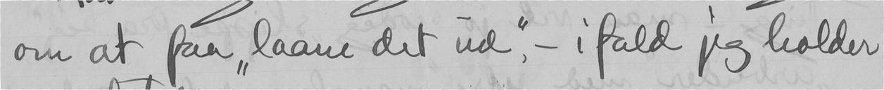om at faa "laane det ud", - i fald jeg holder
